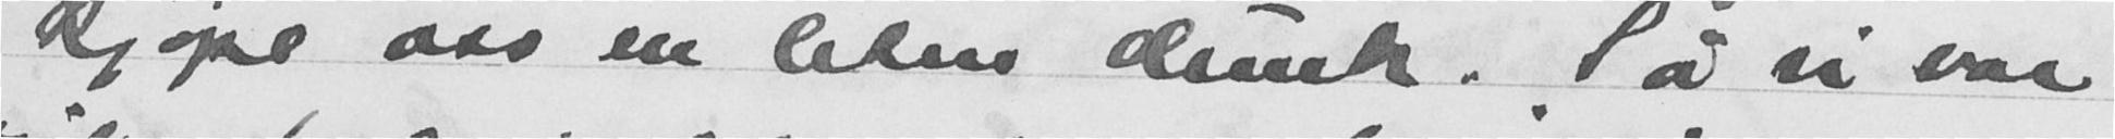kjøpe oss en liten dunk. Så vi var
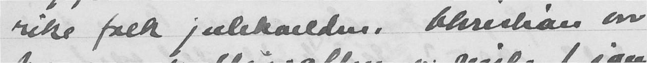rike folk julekvelden. Christian var
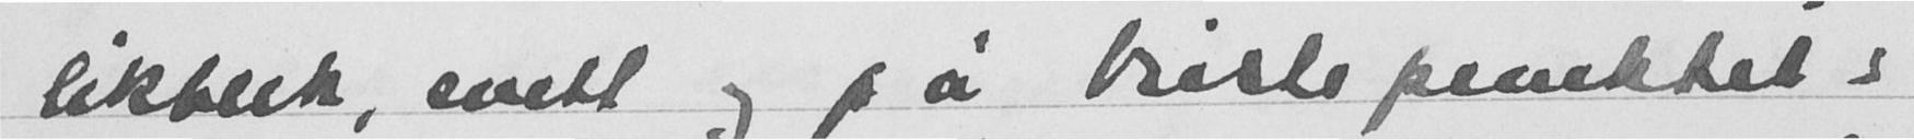likblek, svett og på bristepunktet.
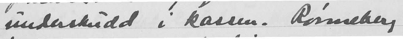underskudd i kassen. Rønneberg
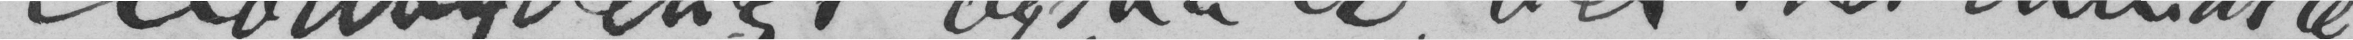modbydeligt ogsaa er, eller i det mindste,
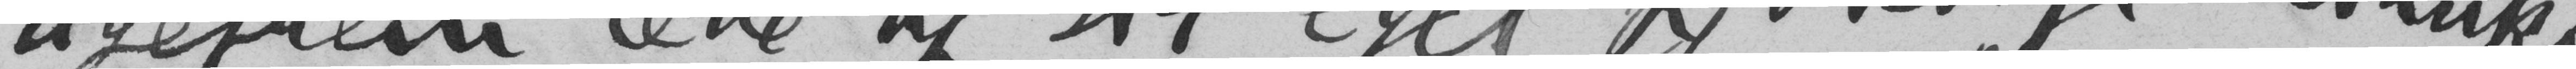ligefrem lede af sit eget kjønslige instinkt,
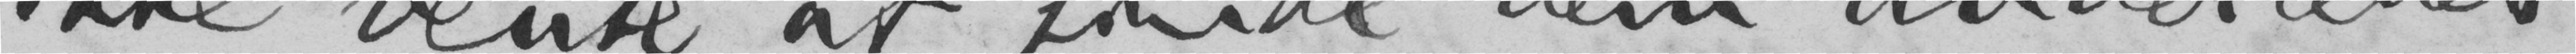ikke vente at finde dem annerledes
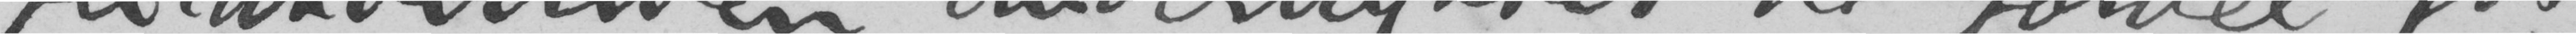fuldkommen undertrykkes til fordel for
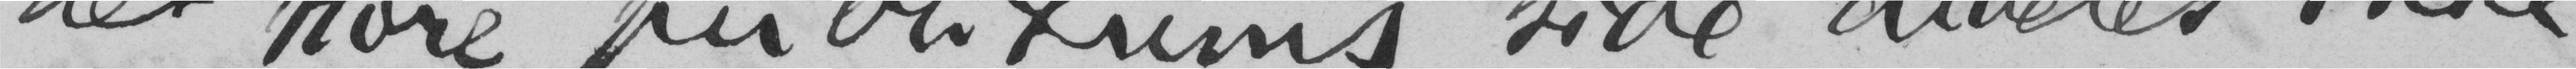det store publikums side aldeles ikke
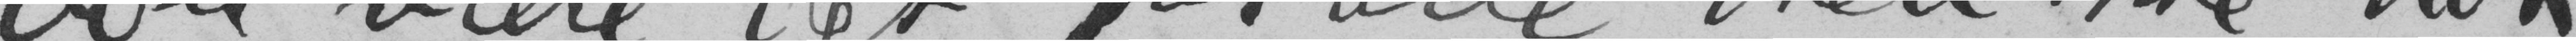bør være det for alle. Men ikke nok
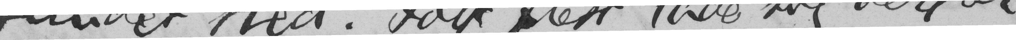fundet sted. Folk flest lade sig derfor
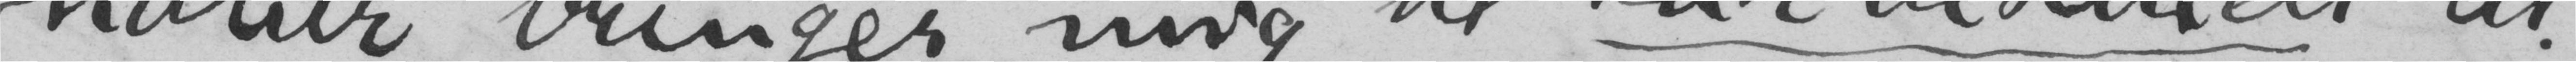natur, bringer mig til intellektuelt at
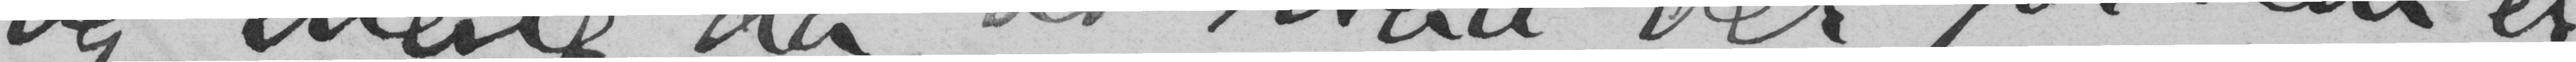og mene da, at hvad der for dem er
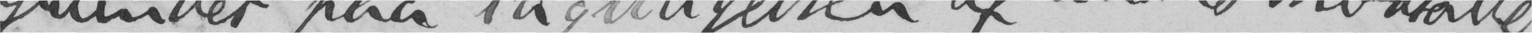grundet paa iagttagelsen af andres modsatte
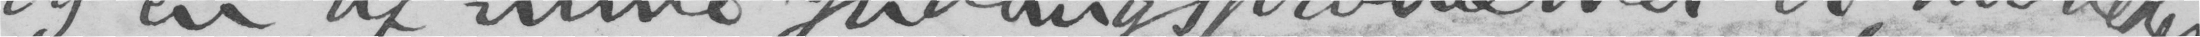og en af mine yndlingsproblemer er, hvorledes
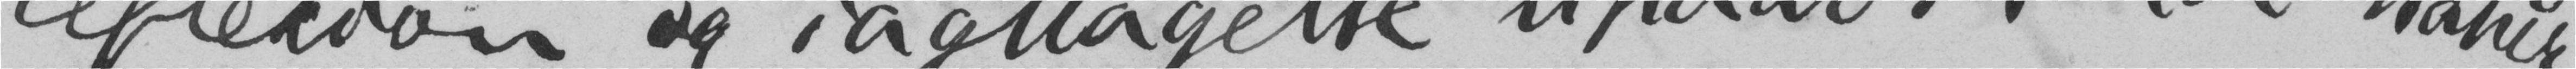reflexion og iaggttagelse upaavirkede natur-
